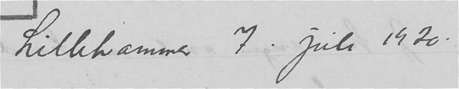Lillehammer 7. juli 1920.
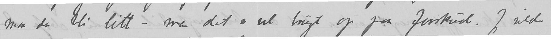maa da bli litt – men det er vel brugt op paa forskud. Jeg vilde
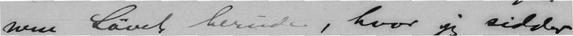nem Løvet herude, hvor jeg sidder,
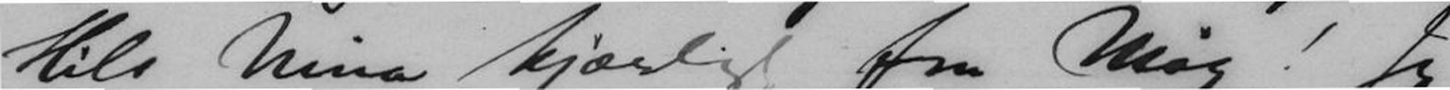Hils Nina kjærligt fra Mig! Jeg
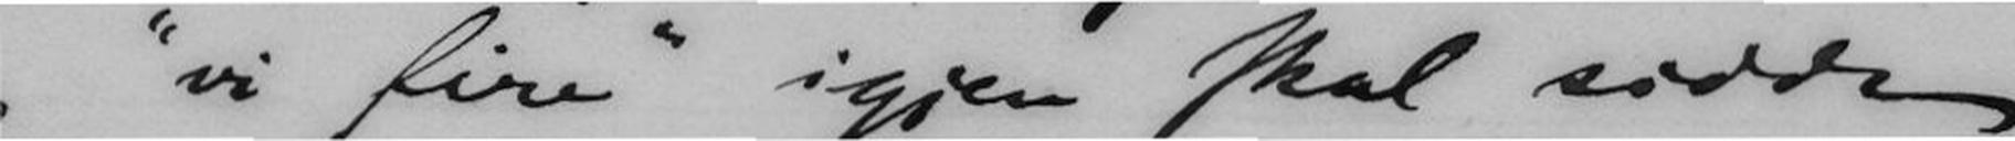og "vi fire" igjen skal sidde
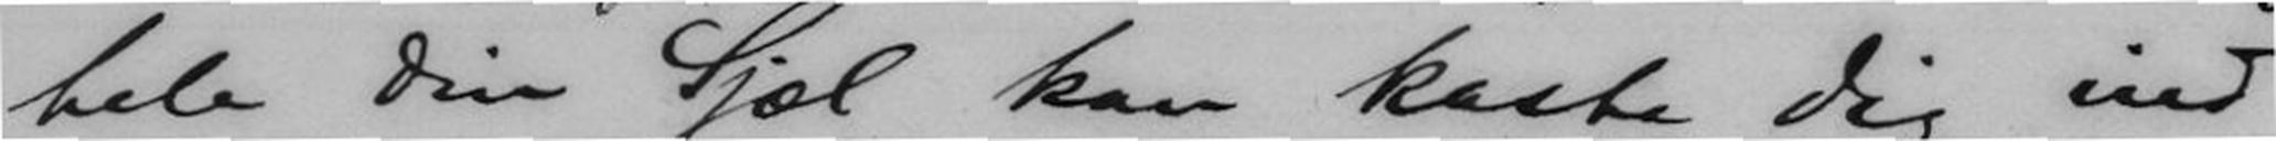hele din Sjæl kan kaste dig ind
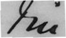Din
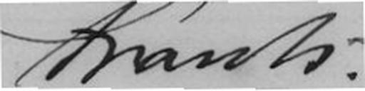Frants
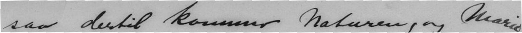saa dertil kommer Naturen, og Marie
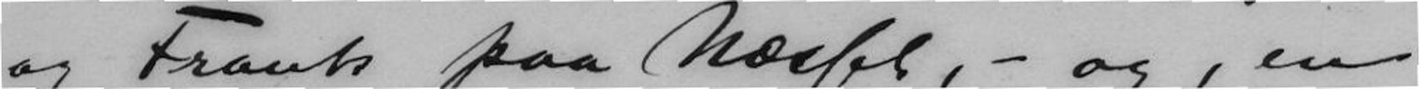og Frants paa Næsset, - og, en
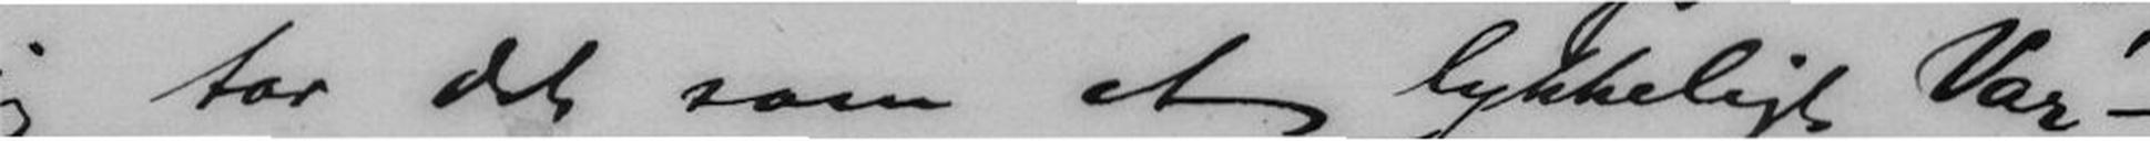jeg tar det som et lykkeligt Var-
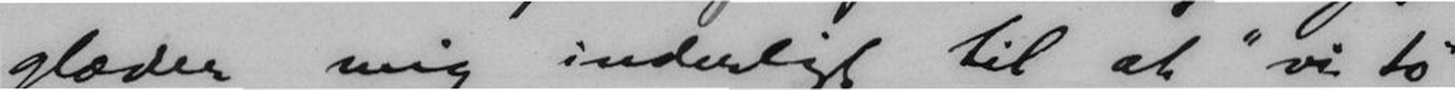glæder mig inderligt til at "vi to"
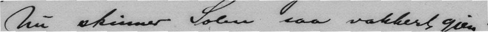Nu skinner Solen saa vakkert gjen-
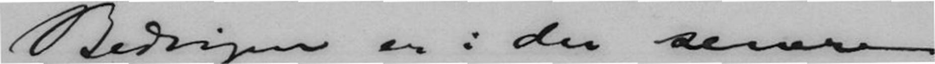Bedringen er i den senere
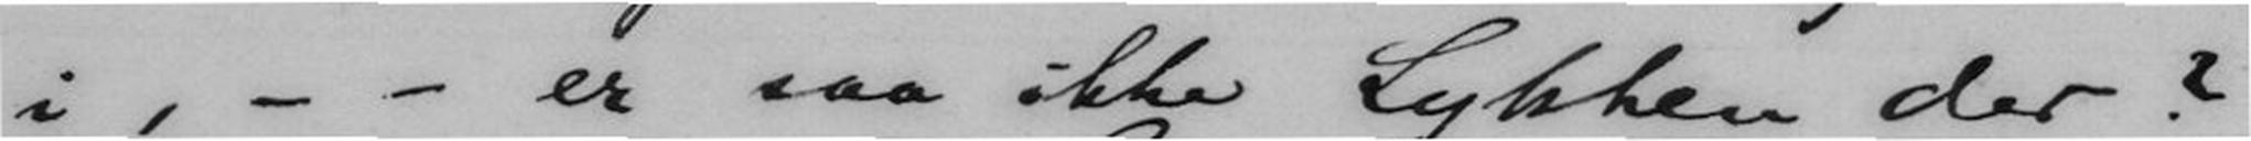i, - - er saa ikke Lykken der?
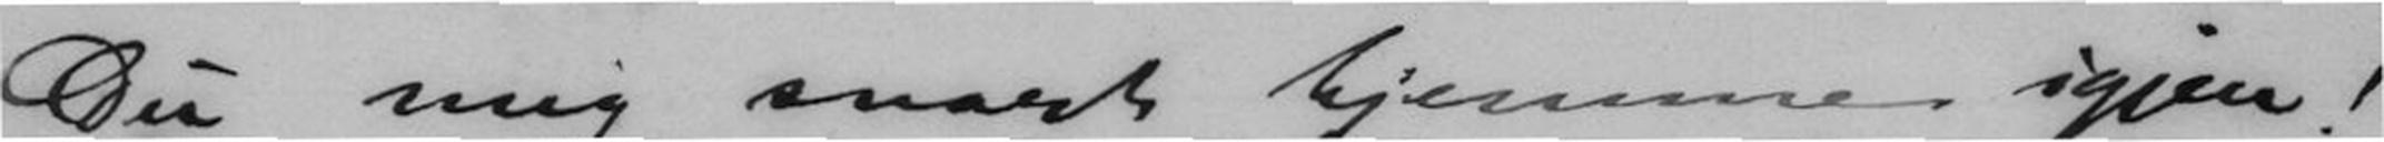Du mig snart hjemme igjen!
